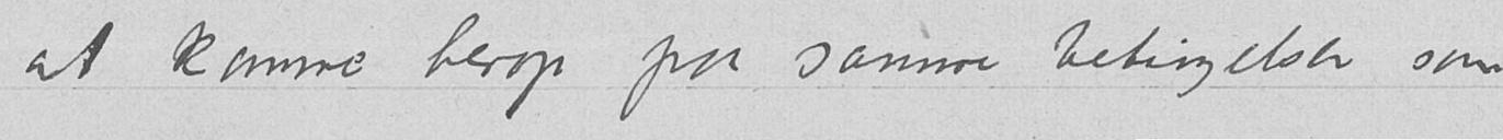at komme herop paa samme betingelser som
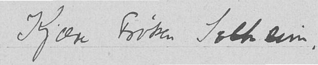Kjære Frøken Solheim,
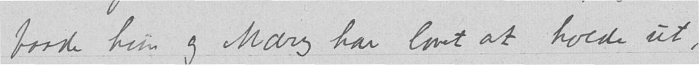baade hun og Mary har lovet at holde ut,
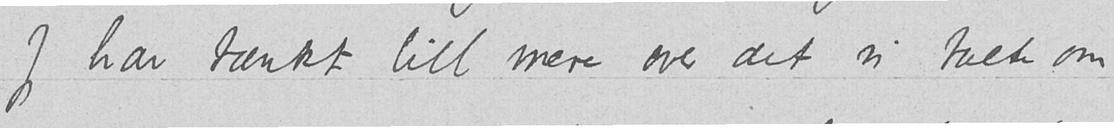Jeg har tænkt litt mere over det vi talte om
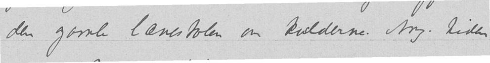den gamle lænestolen om kvelderne. Ang. tiden
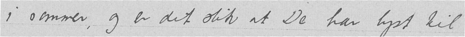i sommer, og er det slik at De har lyst til
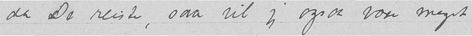da De reiste, saa vil jeg ogsaa være meget
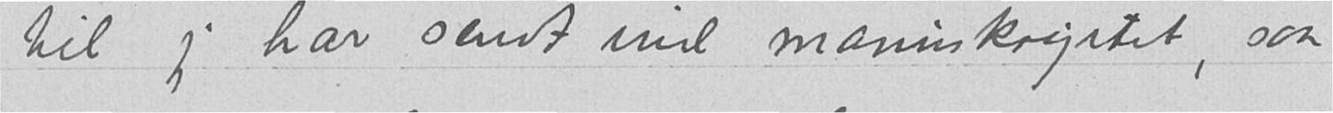til jeg har sendt ind manuskriptet, saa
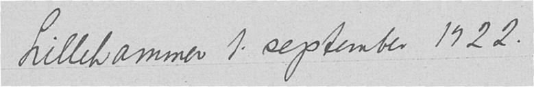Lillehammer 1. september 1922.
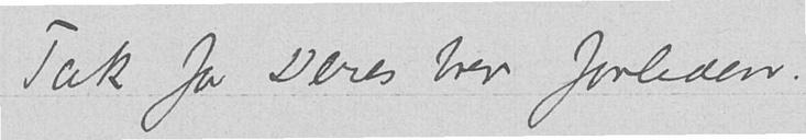Tak for Deres brev forleden.
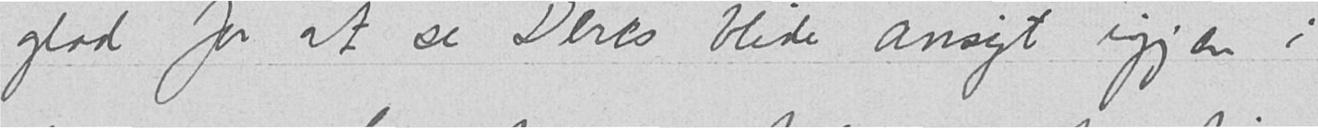glad for at se Deres blide ansigt igjen i
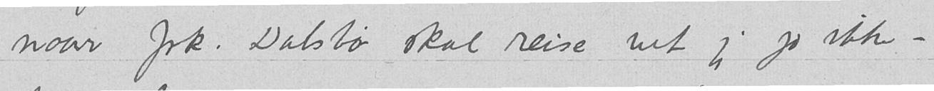naar frk. Dalsbø skal reise vet jeg jo ikke –
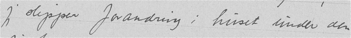jeg slipper forandring i huset under den
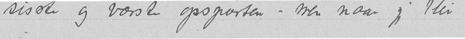sisste og værste opspurten – men naar jeg blir
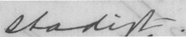stadigt.
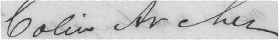Colin Archer
